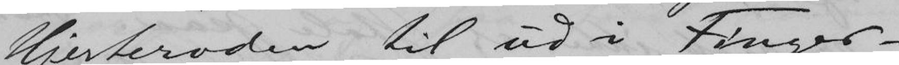Hjerteroden til ud i Finger-
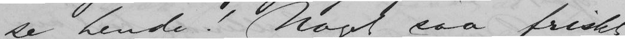se hende! Noget saa friskt,
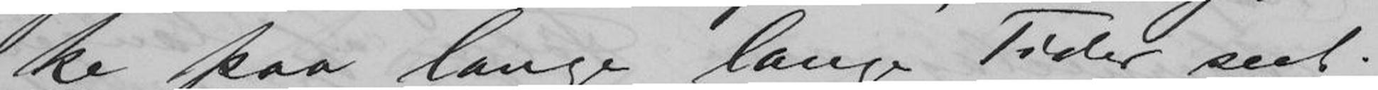ke paa lange lange Tider seet.
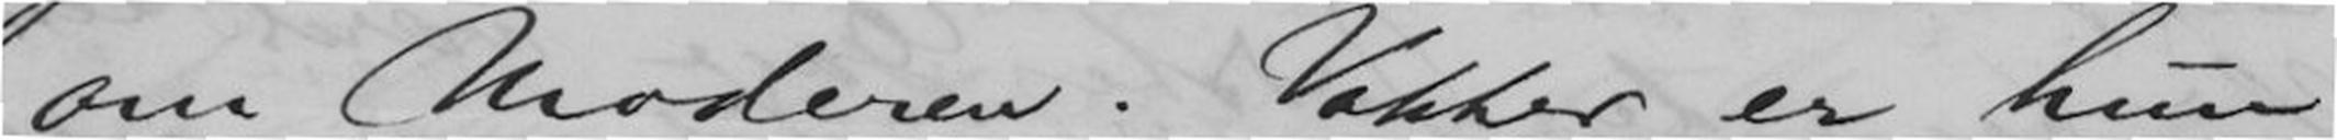om Moderen. Vakker er hun
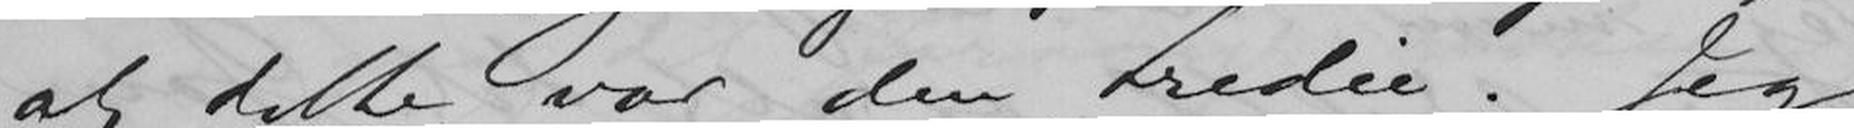at dette var den tredie. Jeg
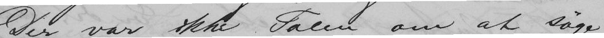Der var ikke Talen om at søge
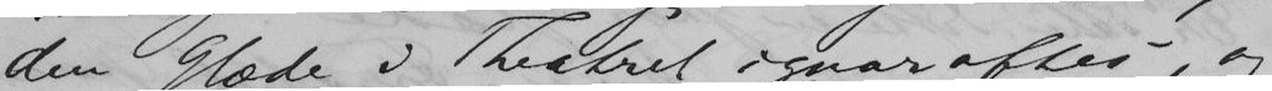den Glæde i Theatret igaaraftes, og
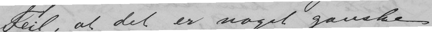Feil, at det er noget ganske
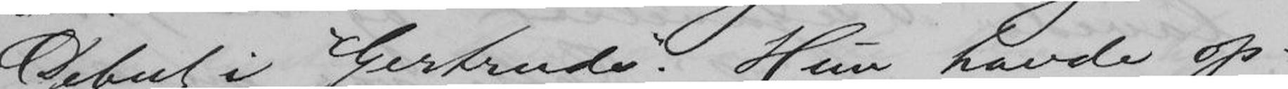Debut i "Gertrude". Hun havde op-
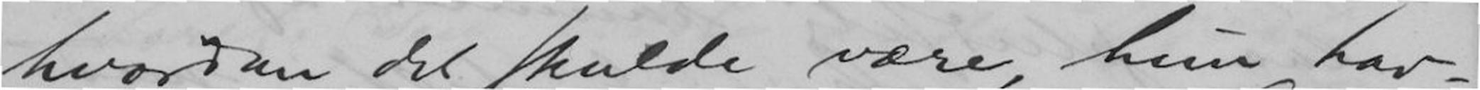hvordan det skulde være, hun hav-
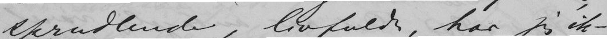sprudlende, livfuldt, har jeg ik-
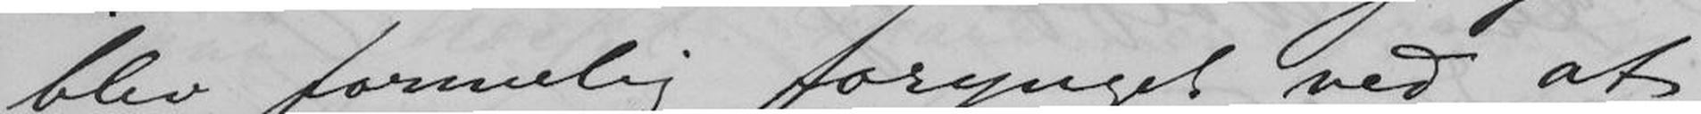blev formelig forynget ved at
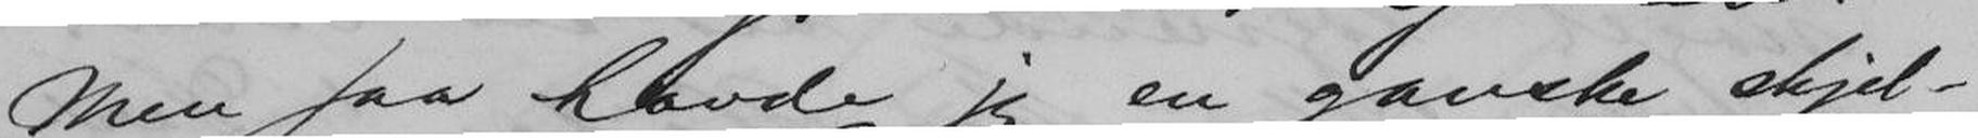Men saa havde jeg en ganske skjel-
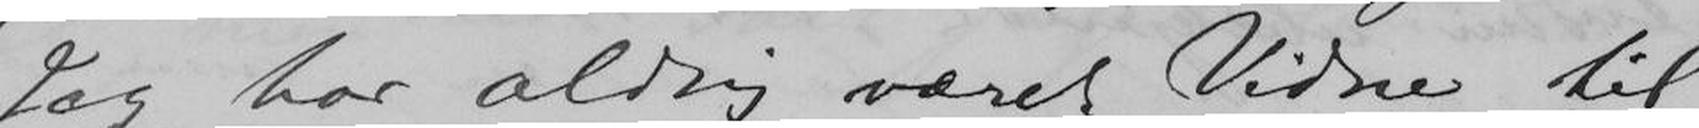Jeg har aldrig været Vidne til
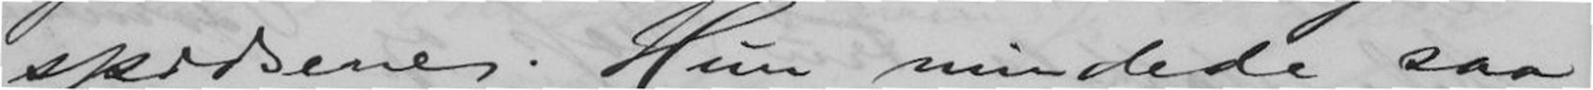spidsene. Hun mindede saa
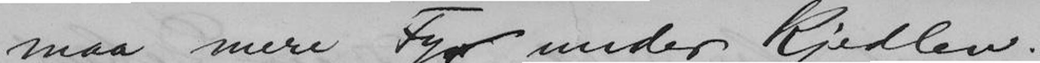maa mere Fyr under Kjedlen.
